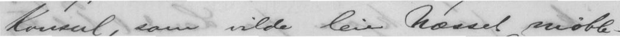Konsul, som vilde leie Næsset møble-
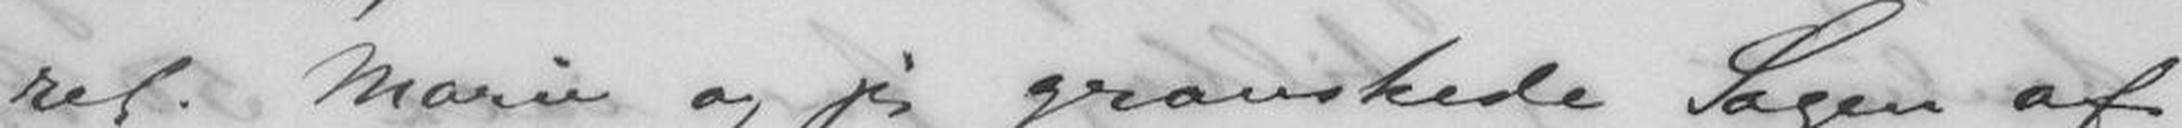ret. Marie og jeg granskede Sagen af
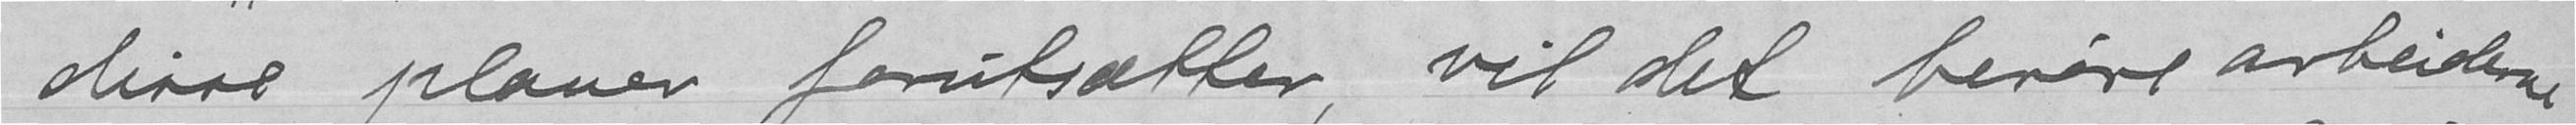disse planer forutsætter, vil det berøre arbeiderne
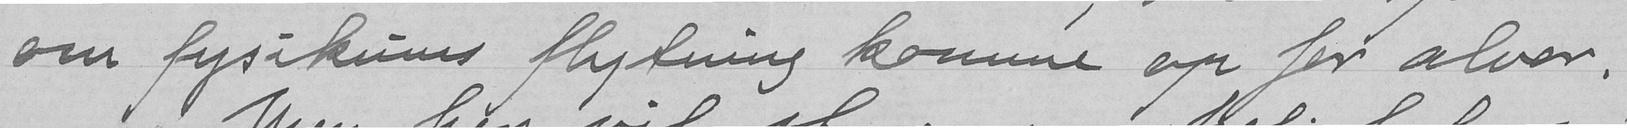om fysikums flytning komme op for alvor.
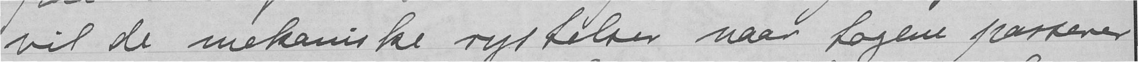vil de mekaniske rystelser naar togene passerer
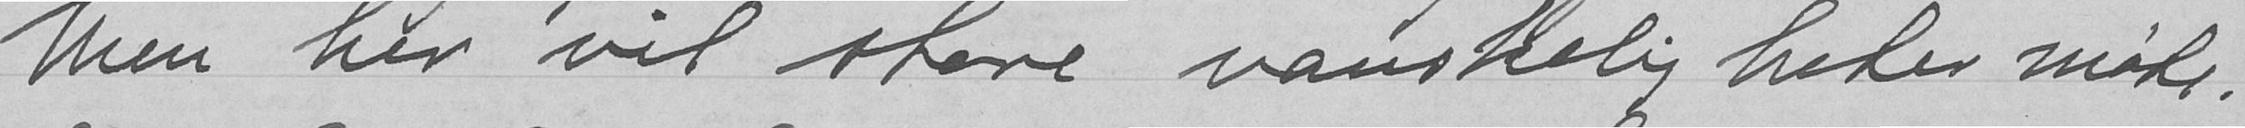Men her vil stere vanskeligheder møte.
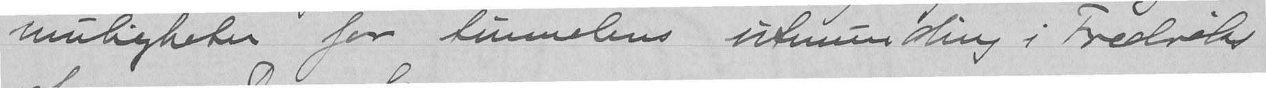muligheder for tunnelens utmunding i Fredriks
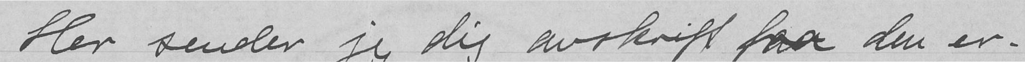Her sender jeg dig avskrift fra den er-
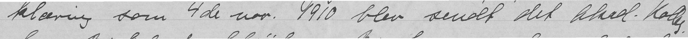klæring som 4de nov. 1910 blev sendt det Akad. Kolleg,
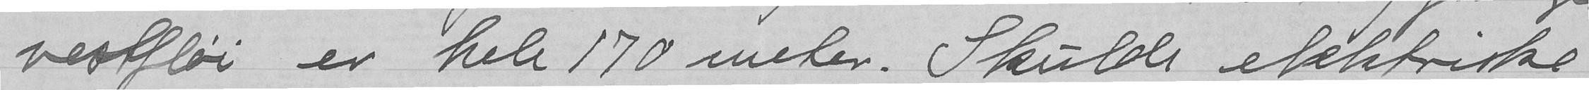vestfløi er hele 170 meter. Skulde elektriske
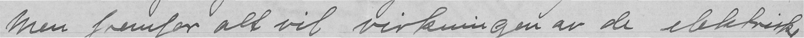Men fremfor alt vil virkningen av de elektriske
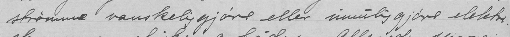strømme vanskeliggjøre eller umuliggjøre elektri-
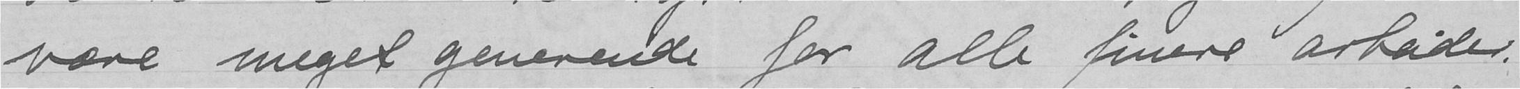være meget generende for alle finere arbeider.
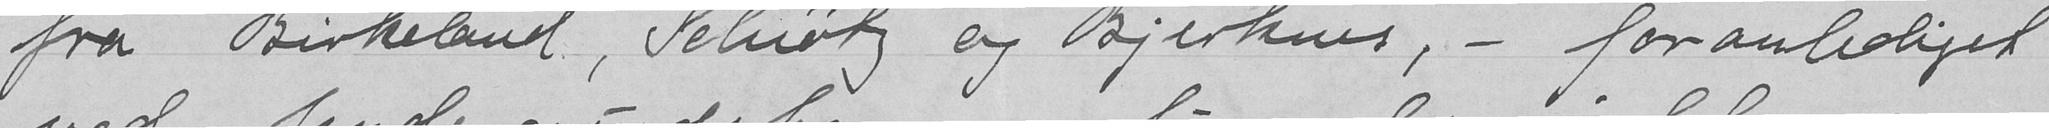fra Birkeland, Schiøtz og Bjerhus, - foranlediget
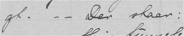gt.- Der staar:
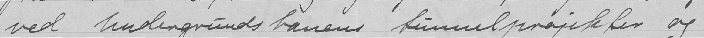ved Undergrundsbanens tunnelprojekter og
